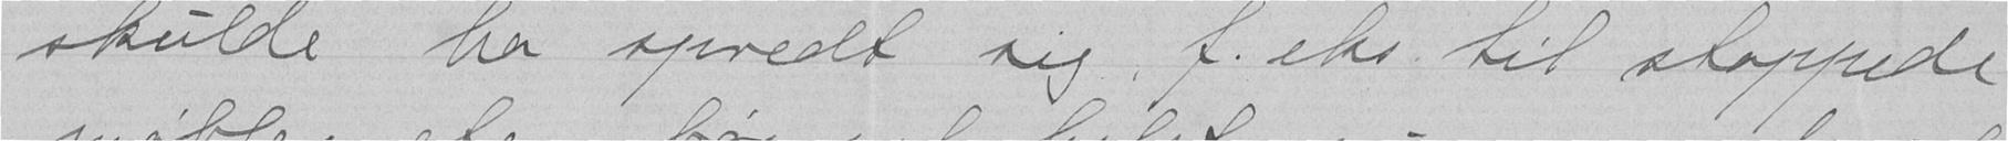skulde ha spredt sig, f.eks. til stoppede
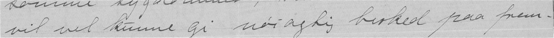vil vel kunne gi nøiagtig besked paa frem-
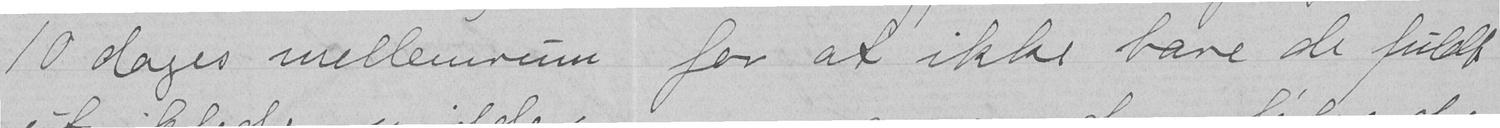10 dages mellemrum for at ikke bare de fuldt
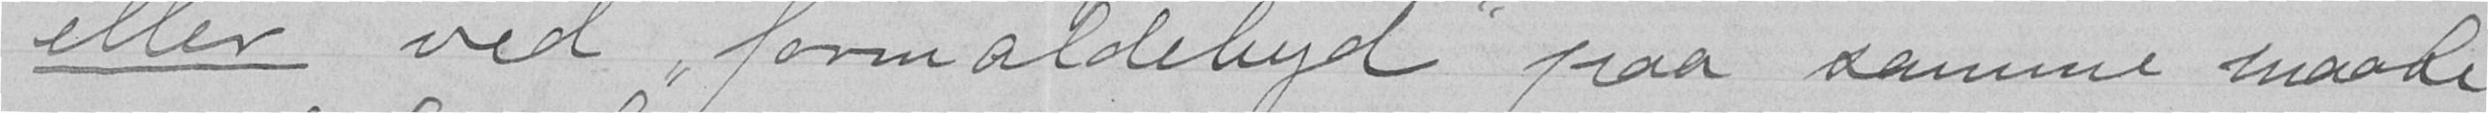eller ved formaldehyd paa samme maate
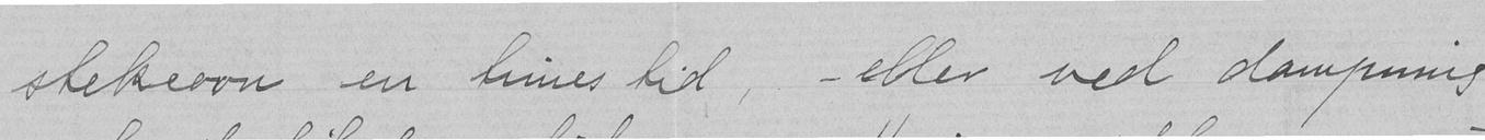stekeovn en times tid, - eller ved dampning
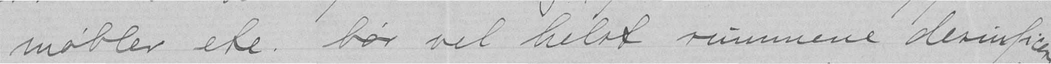møbler ete. bør vel helst rummene desinficeres
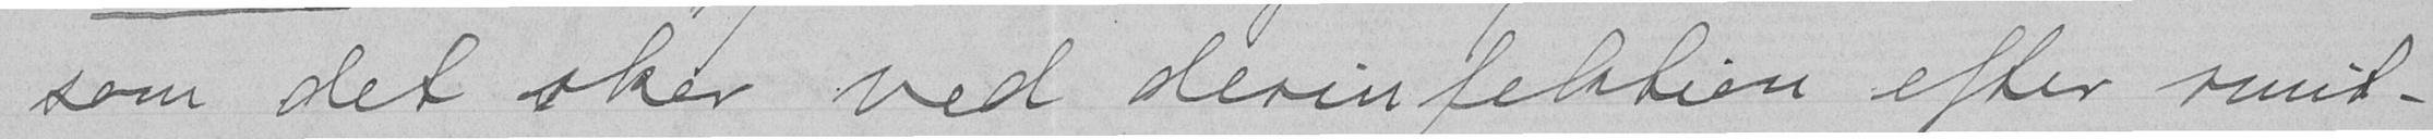som det sker ved derinfektion efter smit-
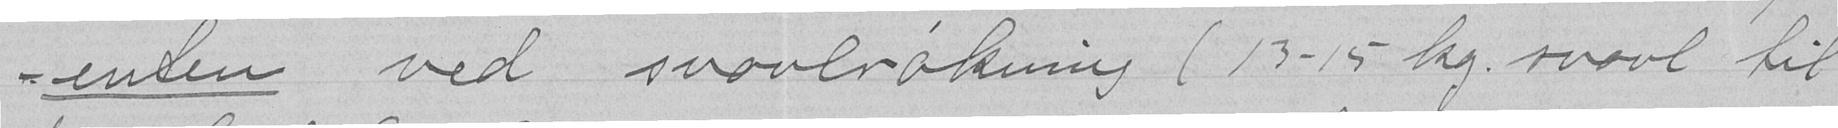- enten ved Svovlrøkning (13-15 kg. svovl til
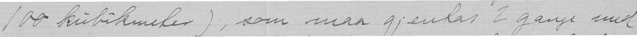100 kubikmeter), som maa gjentas 2 gange med
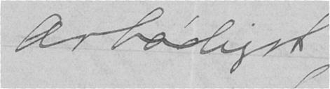Ærbødigst
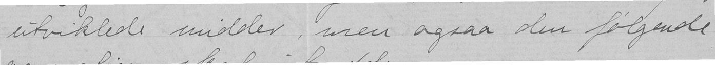utviklede midder, men ogsaa den følgende
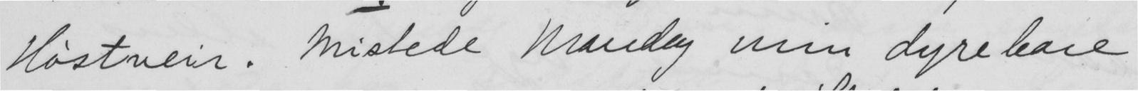Høstveir. Mistede Mandag min dyrebare
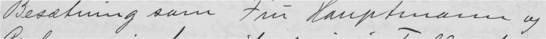Besætning som Fru Hauptmann og
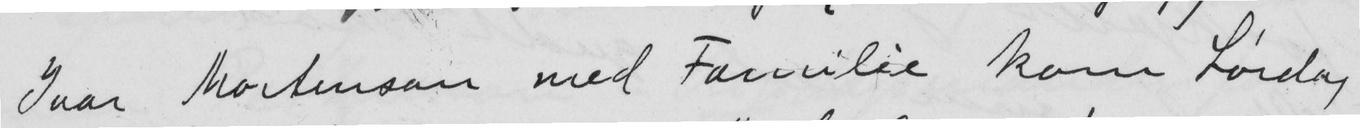Ivar Mortenson med Familie kom Lørdag
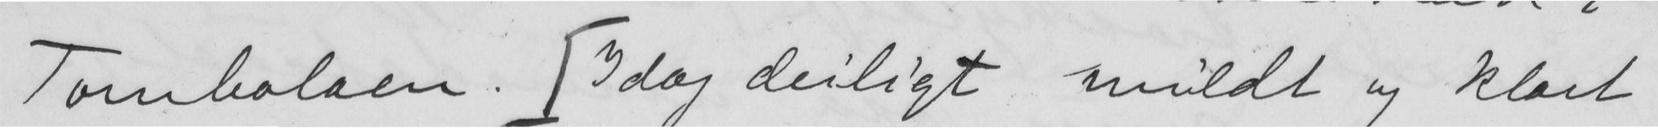Tombolaen. Idag deiligt mildt og klart
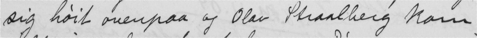sig høit ovenpaa og Olav Straalberg kom
